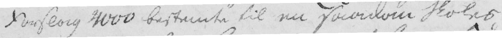Forslag 4000 bestemte til en saadan Skoles
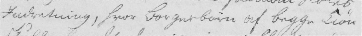Indretning, hvor Borgerbørn af begge Kiøn
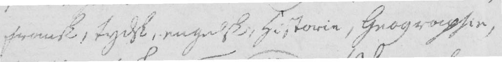fransk, tydsk, engelsk, historie, Geographie,
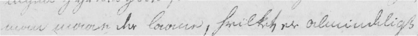man maae der laane, hvilket er almindeligt
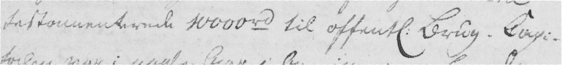testamenterede 10000 rd til offentl. Brug. Capi-
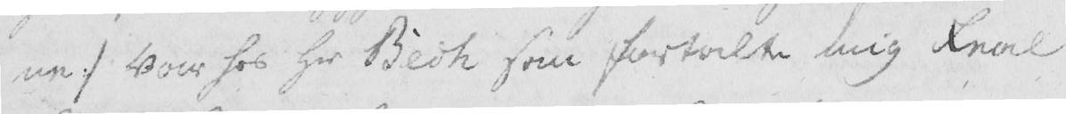ne :/ var hos Hr Bech som fortalte mig Real
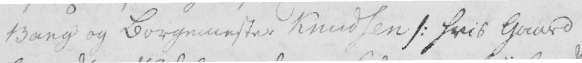Bang og Borgemester Knudsen /: hvis Gaard
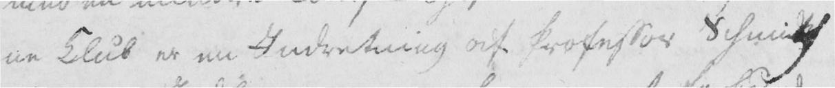ne Klub er en Indretning af Professor Schmidt
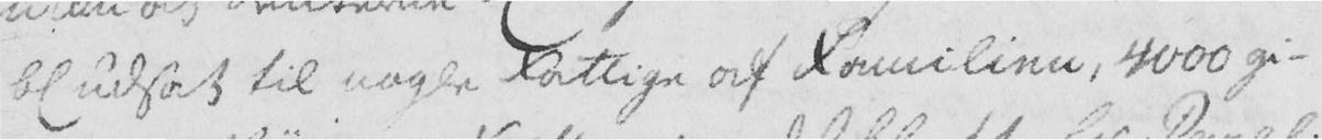bl udsat til nogle Fattige af Familien, 4000 gi-
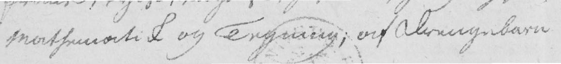Mathematik og Tegning; af Drengebarn
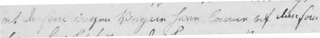at de som ingen Vogne have laane af dem som
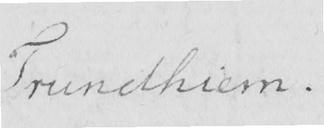Trundhiem.
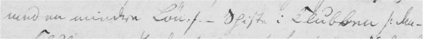med en mindre Løn :/. - Spiste i Klubben /: den-
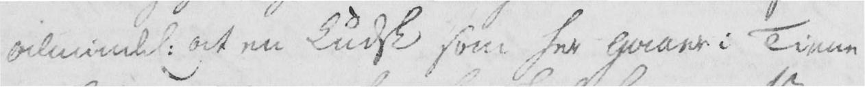almindel. at en Kudsk som her gaaer i Tiene-
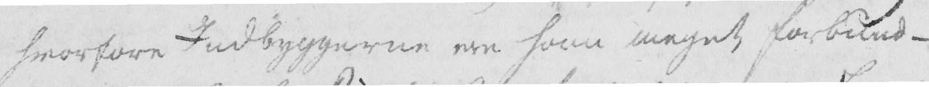hvorfore Indbyggerne ere ham meget forbund-
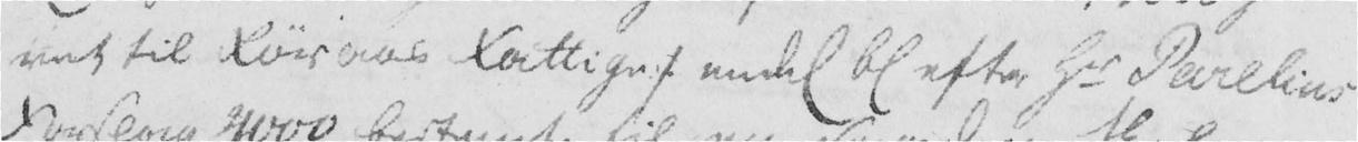vet til Røraas Fattige :/ endel bl efter Hr Parelius
